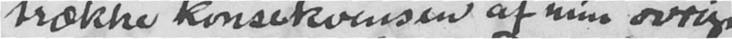trække konsekvensen af min øvrgie
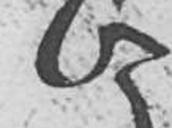Og
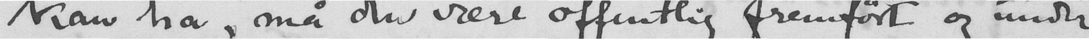kan ha, må den være offentlig fremført og under
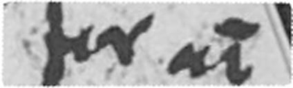for at
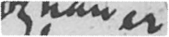og han er
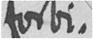forbi.
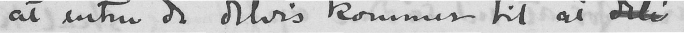at enten de delvis kommer til at dele
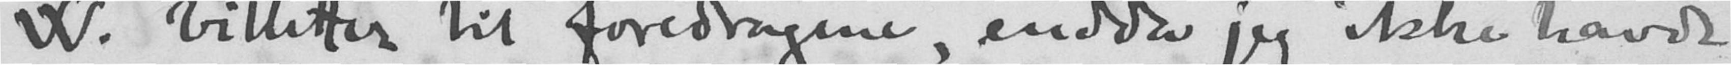W. billetter til foredragene, endda jeg ikke havde
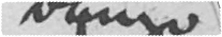bange
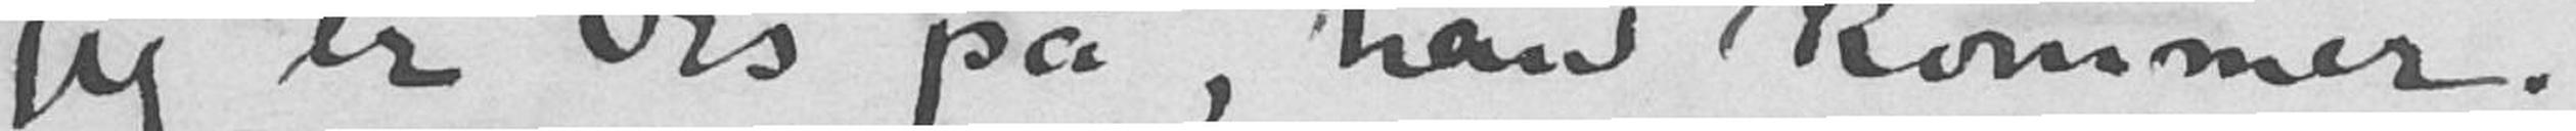jeg er vis på, han kommer.
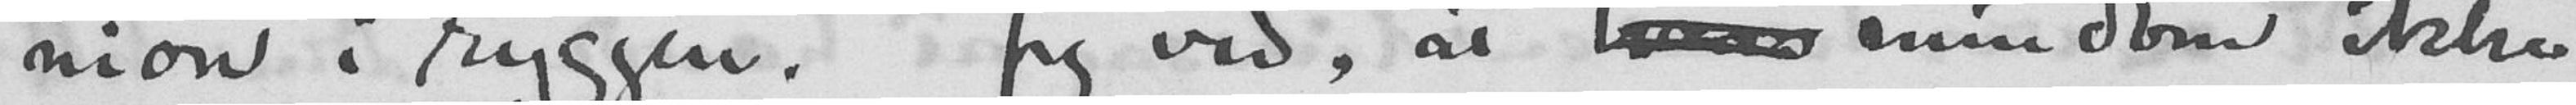nion i ryggen. Jeg ved, at hans min dom ikke
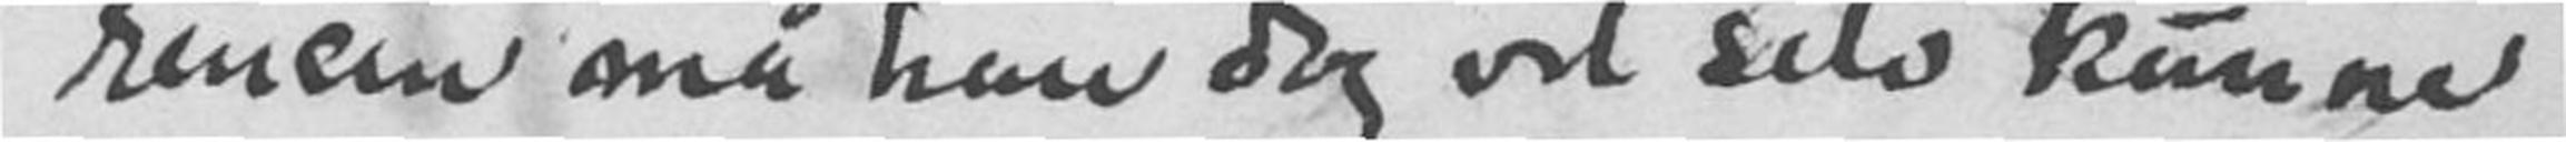rencen må han dog vel selv kunne
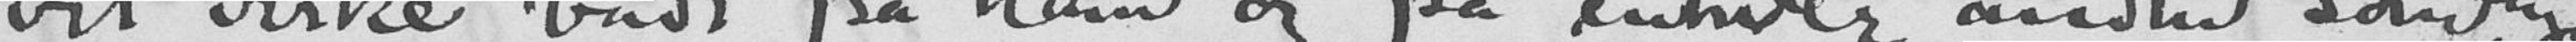vil virke både på ham og på enhver anden som
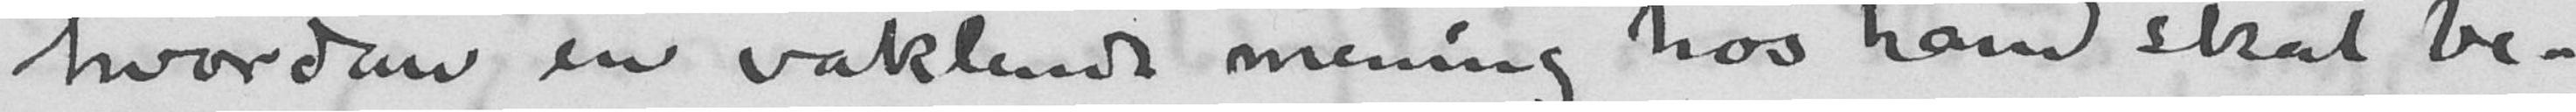hvordan en vaklende mening hos ham skal be-
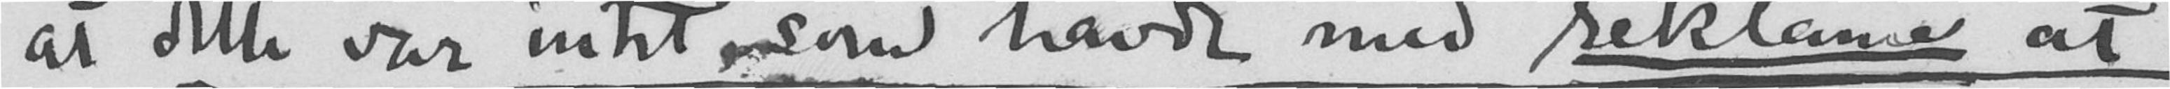at dette var intet som havde med reklame at
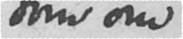dom om
